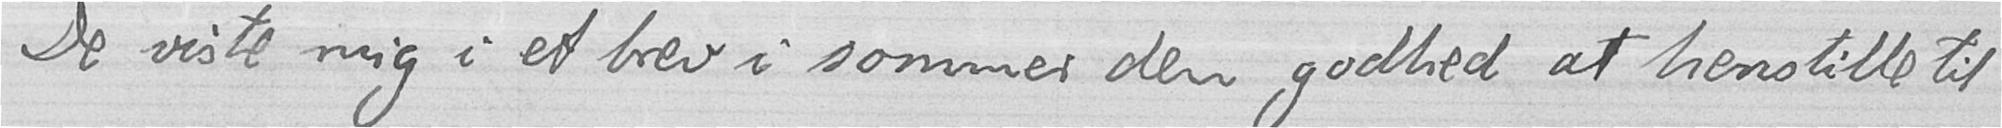De viste mig i et brev i sommer den godhed at henstille til
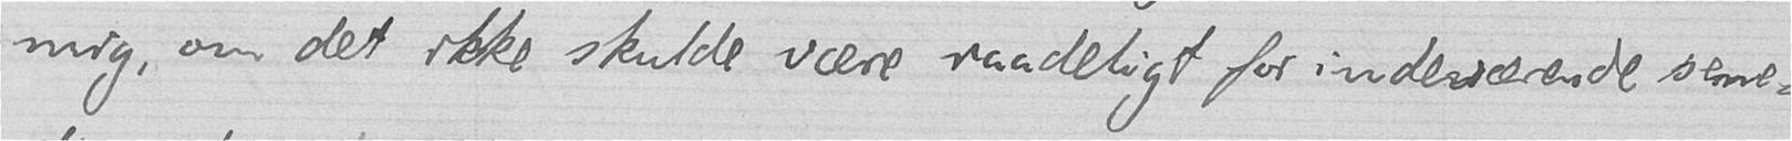mig, om det ikke skulde være raadeligt for indeværende seme-
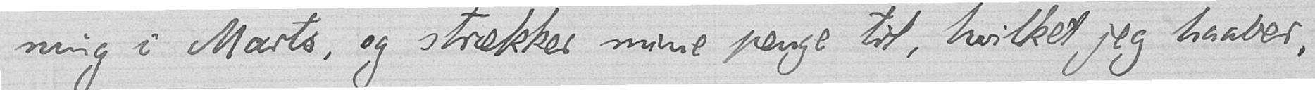ning i Marts, og strækker mine penge til, hvilket jeg haaber,
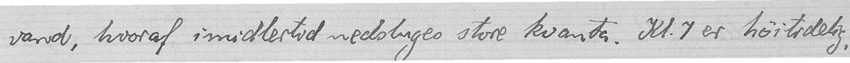vand, hvoraf imidlertid nedsluges store kvanta. Kl. 7 er høitidelig-
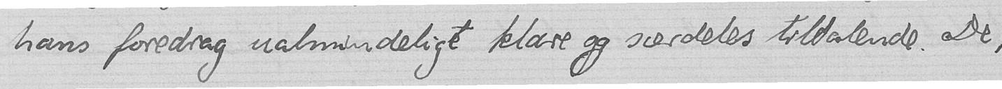hans foredrag ualmindeligt klare og særdeles tiltalende. De,
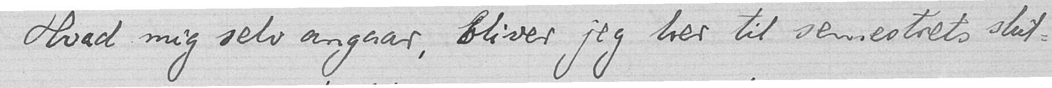Hvad mig selv angaar, bliver jeg her til semestrets slut-
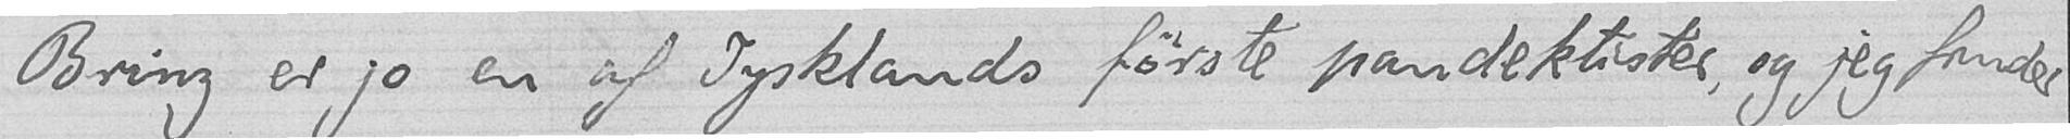Brinz er jo en af Tysklands første pandektister, og jeg finder
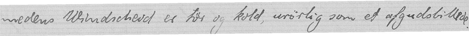medens Windscheid er tør og kold, urørlig som et afgudsbillede.
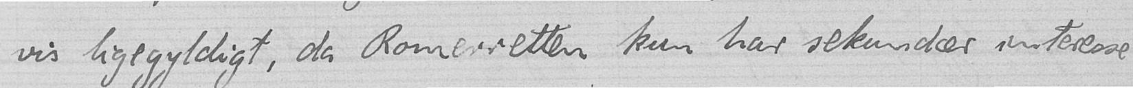vis ligegyldigt, da Romerretten kun har sekundær interesse
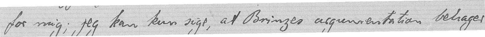for mig; jeg kan kun sige, at Brinzes argumentation behager
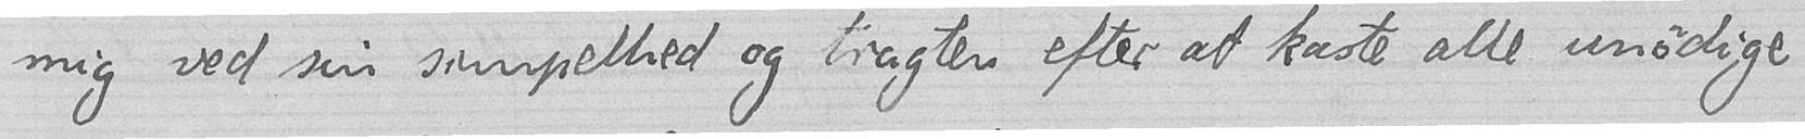mig ved sin simpelhed og tragten efter at kaste alle unødige
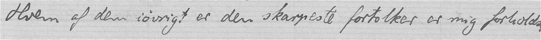Hvem af dem iøvrigt er den skarpeste fortolker er mig forholds-
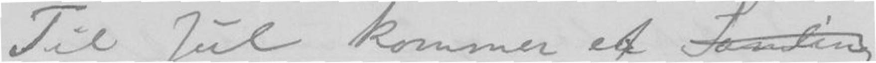Til Jul kommer et Samling
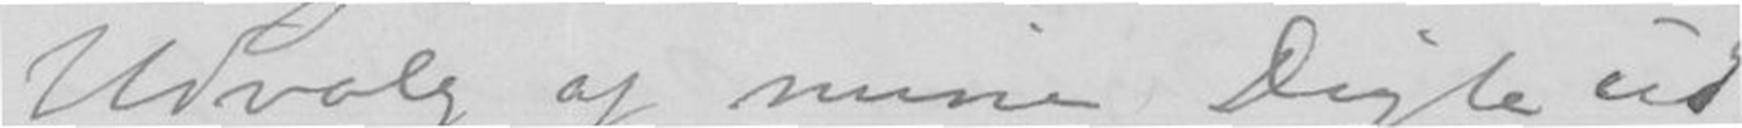Udvalg af mine Digte ud
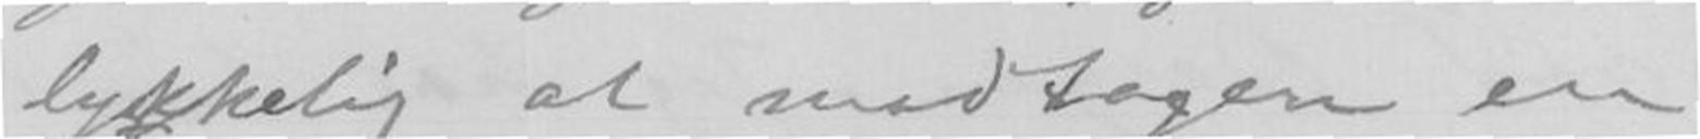lykkelig at modtagen en
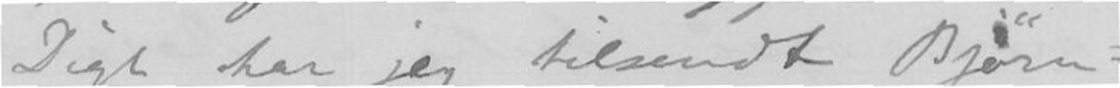har jeg tilsendt Bjørn
